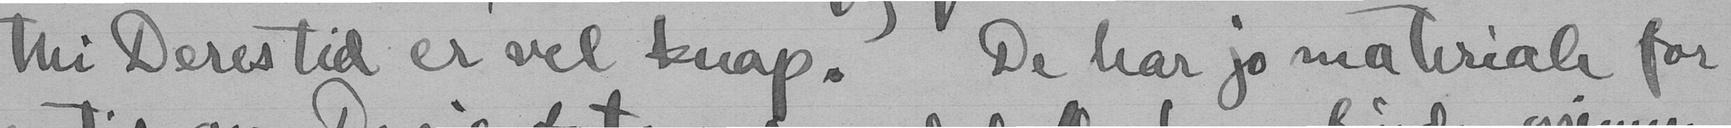thi Deres tid er vel knap. De har jo materiale for
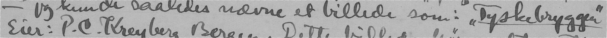-jeg kunde saaledes nævne et billede som : "Tyskebryggen"
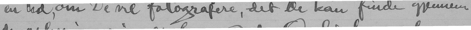en tid, om De vil fotografere, det de kan finde gjennem
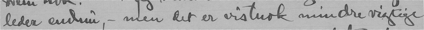leder endnu, - men det er vistnok mindre vigtige
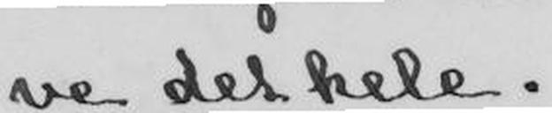ve det hele.
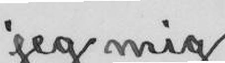jeg mig
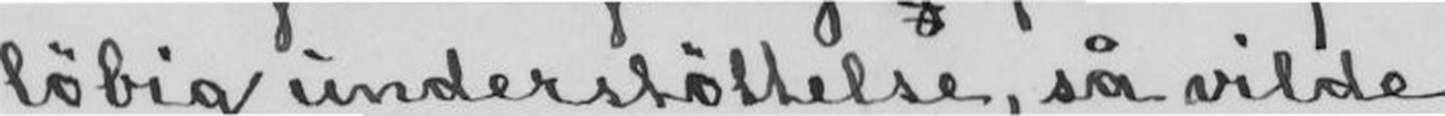løbig understøttelse, så vilde
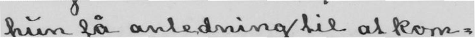hun få anledning til at kom-
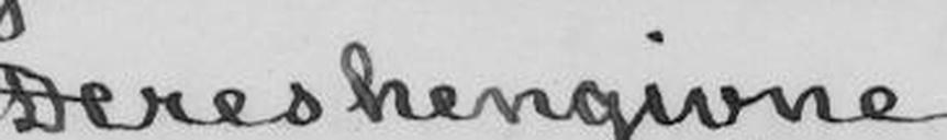Deres hengivne
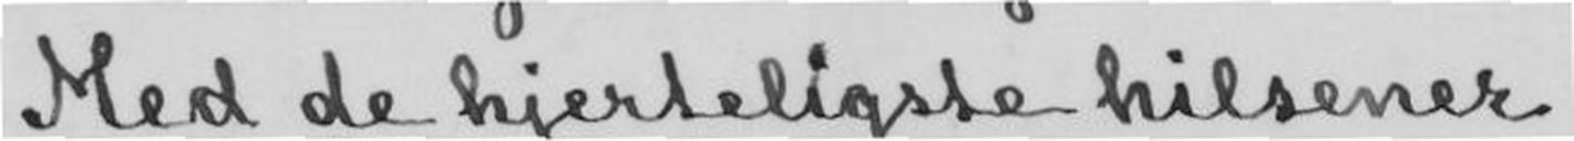Med de hjerteligste hilsener
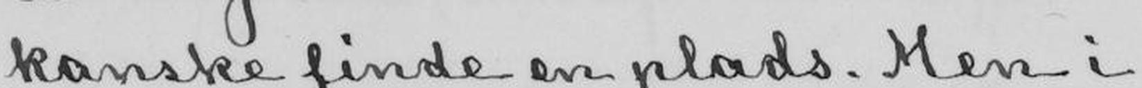kanske finde en plads. Men i
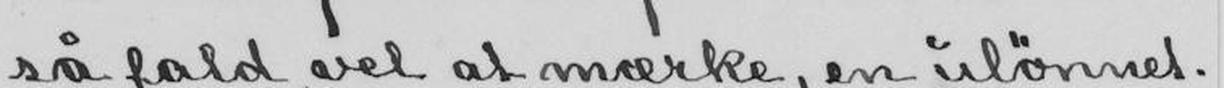så fald vel at mærke, en ulønnet.
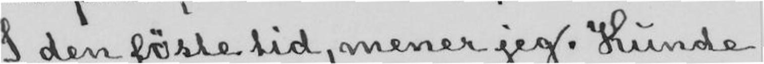I den første tid, mener jeg. Kunde
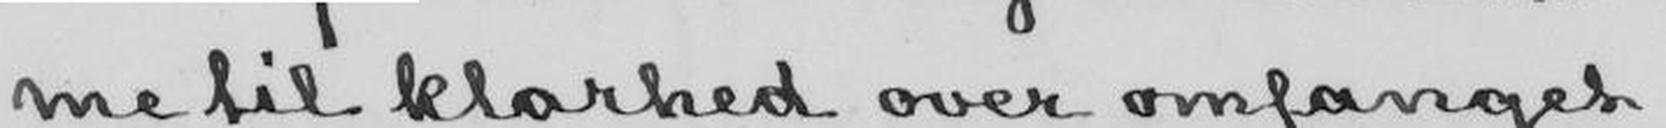me til klarhed over omfanget
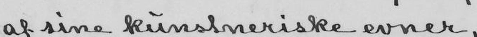af sine kunstneriske evner,
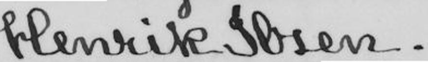Henrik Ibsen.
